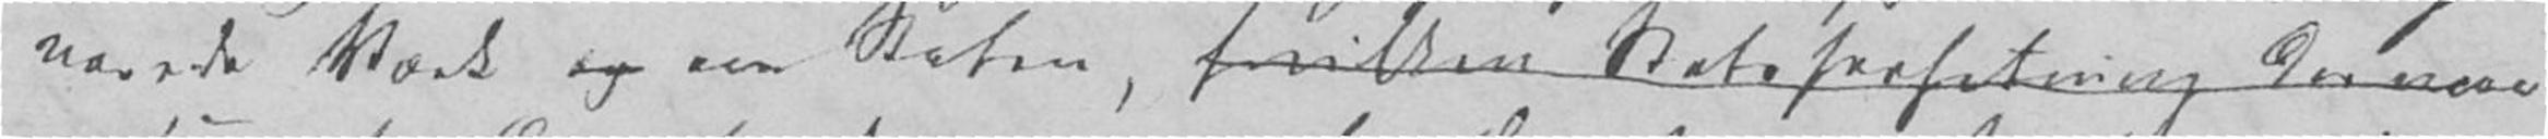varede Værk og om Staten, hvilken Statsforfatning der maa
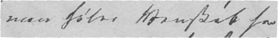man føler Venskab for
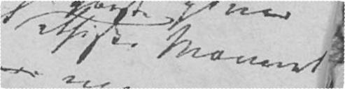ethiske Moment
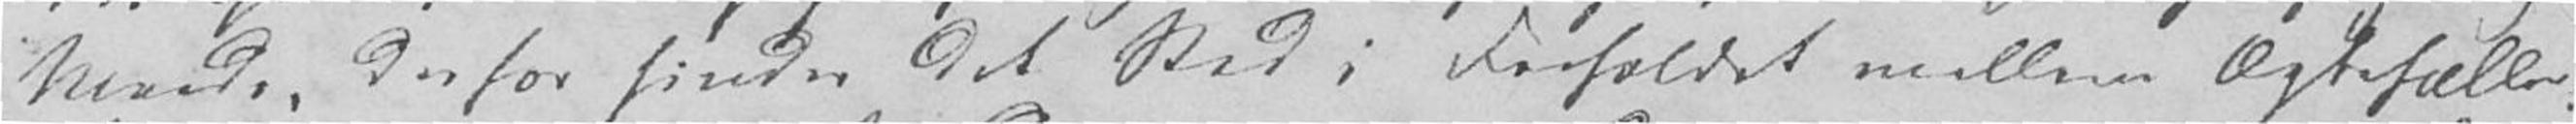Maade. Derfor finder det Sted i Forholdet mellem Ægtefæller,
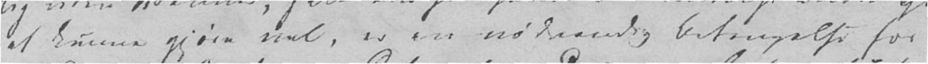at kunne gjøre vel, er en nødvendig Betingelse for
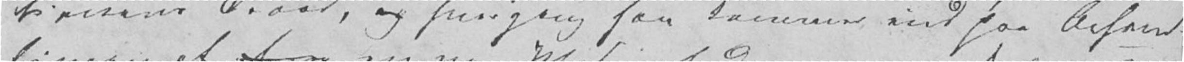tionens Traad, og hvergang han kommer ind paa Behand-
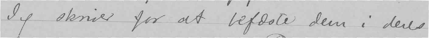Jeg skriver for at befæste dem i deres
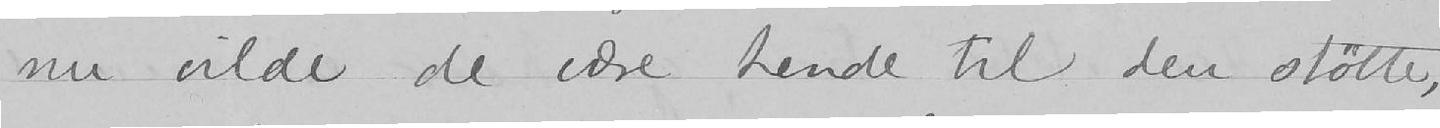nu vilde de være hende til den støtte,
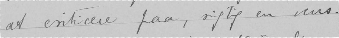at criticere paa, rigtig en vens.
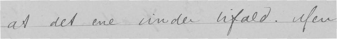at det ene vinder bifald. Men
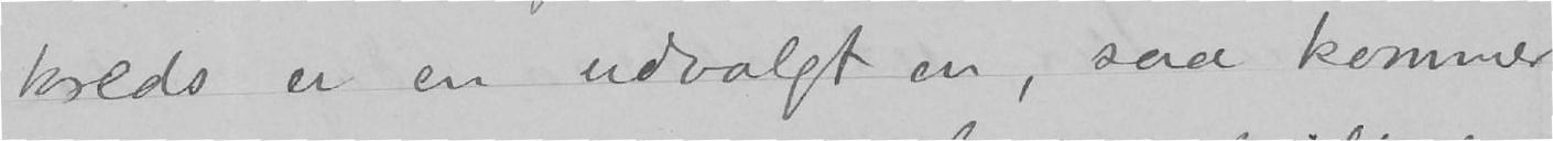kreds er en udvalgt en, saa kommer
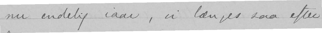nu endelig iaar, vi længes saa efter
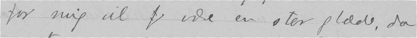for mig vil f være en stor glæde, da
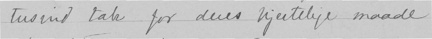tusind tak for deres hjertelige maade
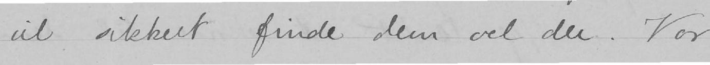vil sikkert finde dem vel der. Vor
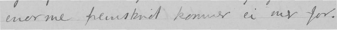enorme fremskridt kommer ei mer for.
